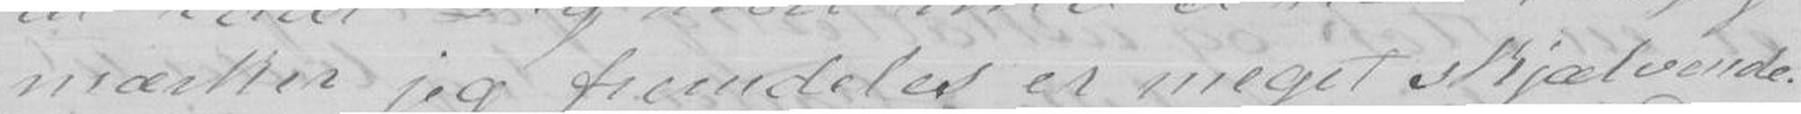mærker jeg fremdeles er meget skjælvende.
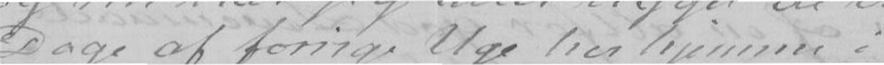Dage af forrige Uge her hjemme i
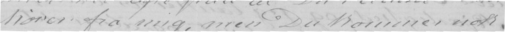hører fra mig, men Du kommer nok
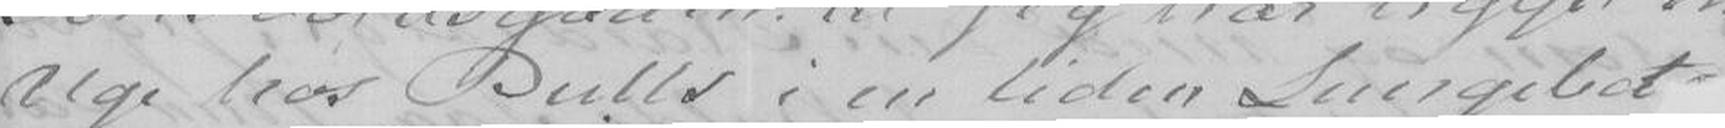Uge hos Bulls i en liden Lungebet
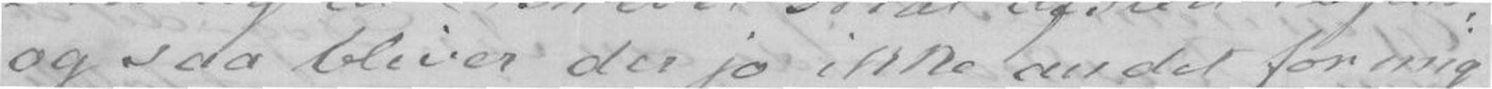og saa bliver der jo ikke andet for mig
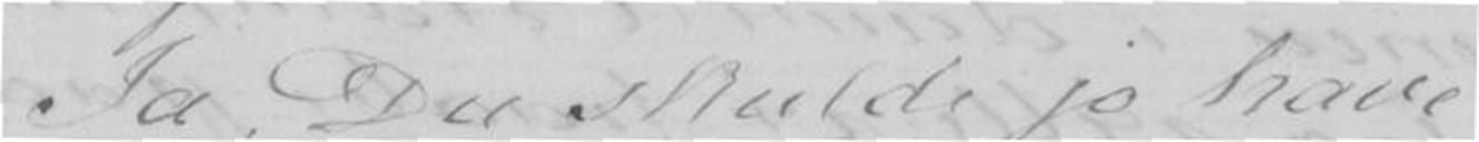Ja Du skulde jo have
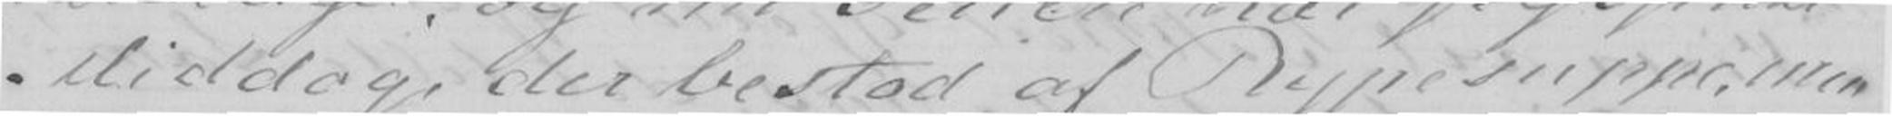Middag der bestod af Rypesuppe, men
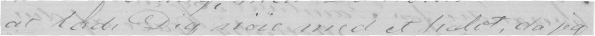at lade Dig nøie med et halvt, da jeg
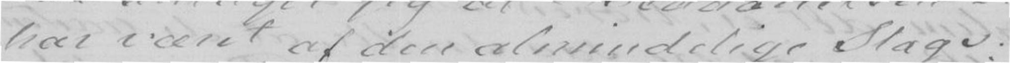har været af den almindelige Slags.
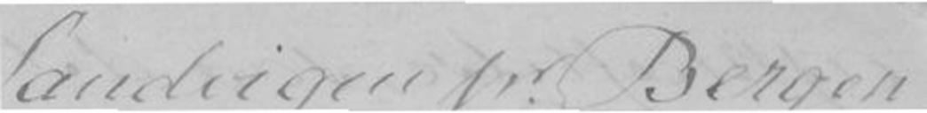Sandvigen pr. Bergen
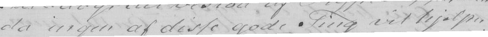da ingen af disse gode Ting vil hjælpe,
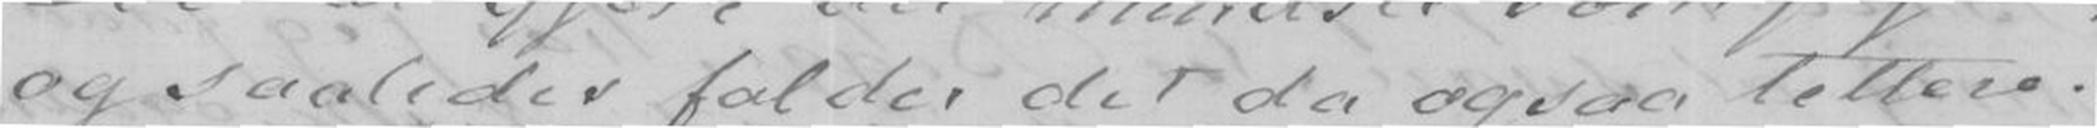og saaledes falder det da ogsaa lettere.
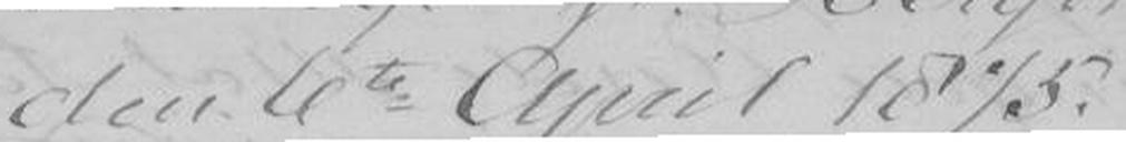den 6te April 1875.
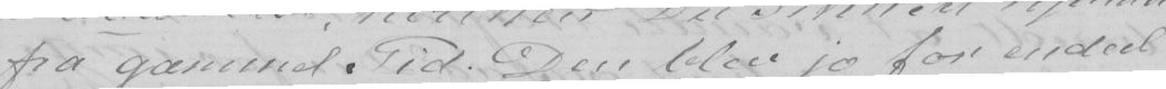fra gammel Tid. Den blev jo for endeel
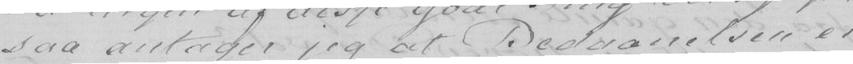saa antager jeg at Bedaanelsen ei
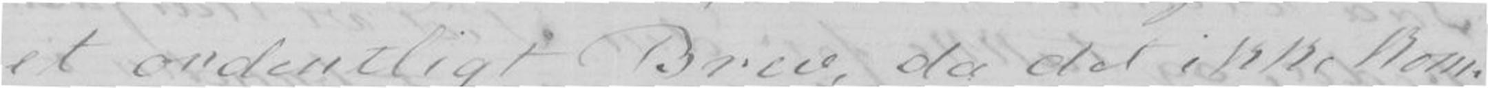et ordentligt Brev, da det ikke kom-
